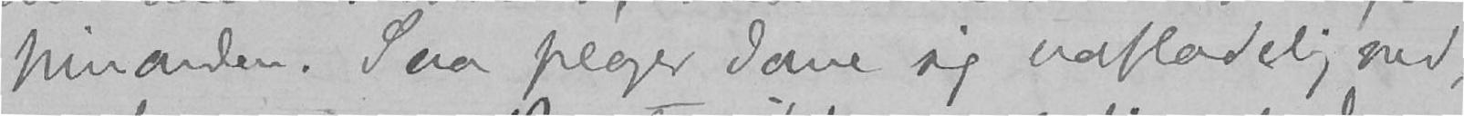hinanden. Saa plager Jane sig uafladelig med,
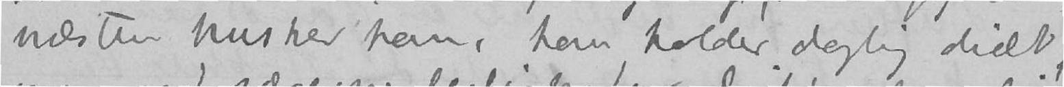næsten husker han, han holder daglig diæt,
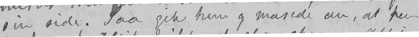sin side. Saa gik hun og masede om, at hun
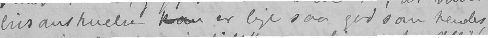livsanskuelse kan er lige saa god som hendes,
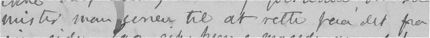mister man evnen til at rette paa det fra
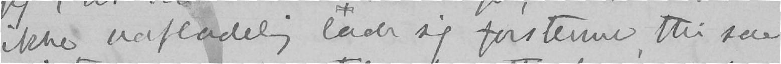ikke uadfladelig lade sig forstemme, thi saa
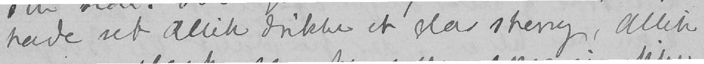havde set Allik drikke et glas sherry, Allik
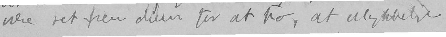være ret frem dum for at tro, at ulykkelige
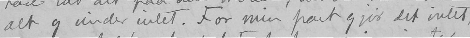alt og vinder intet. For min part gjør det intet,
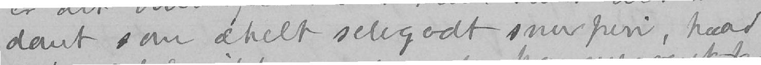dant som ækelt selvgodt snurperi, hvad
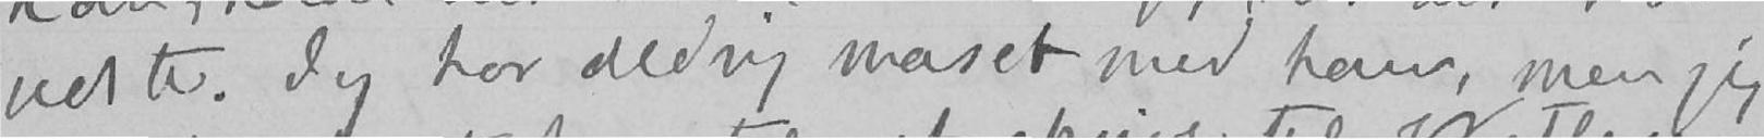bedste. Jeg har aldrig maset med ham, men jeg
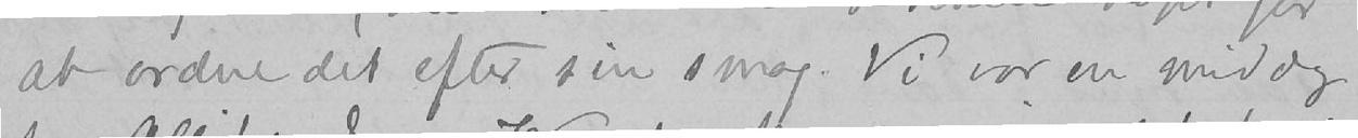at ordne det efter sin smag. Vi var en middag
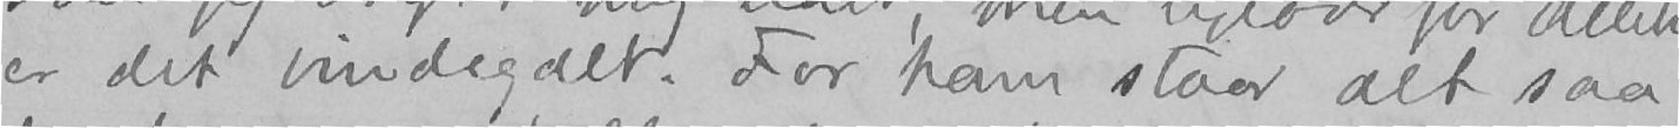er det bindegalt For ham staar alt saa-
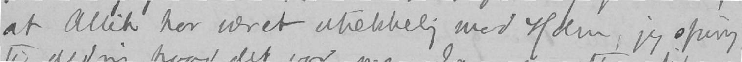at Allik har været utekkelig med Holm, jeg spurg-
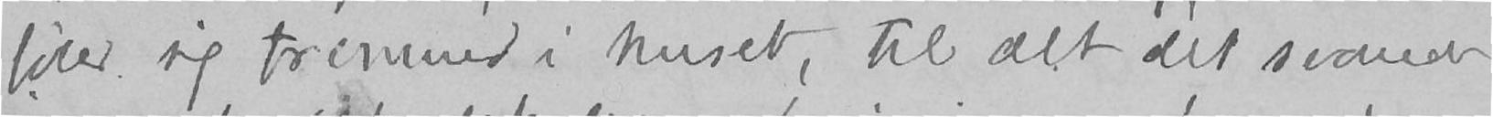føler sig fremmed i huset, til alt det svarede
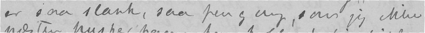er saa slank, saa pen og ung, som jeg ikke
